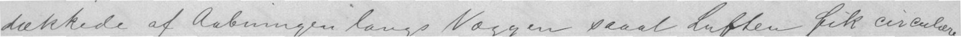dækkede af Aabninger langs Væggen saaat luften fik circulere
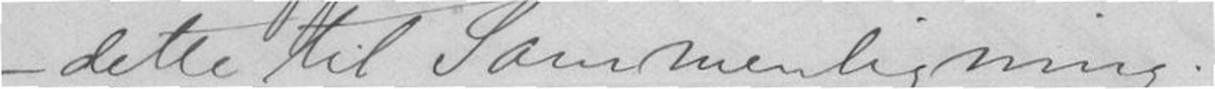- dette til Sammenligning.
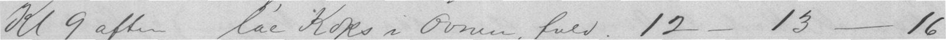Kl 9 aften làe Koks i Ovnen, fuld12 - 13 - 16
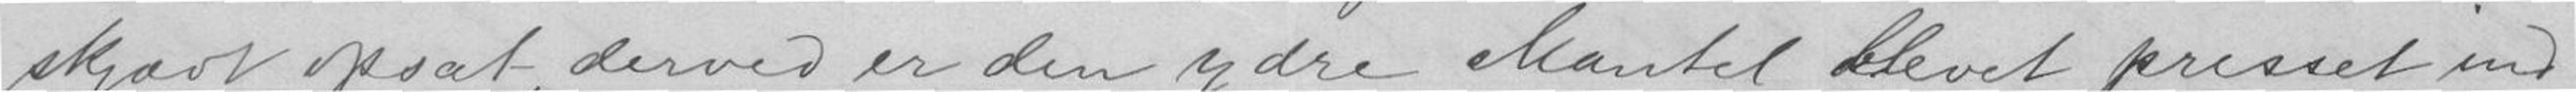skjævt opsat, derved er den ydre Mantel blevet presset ind
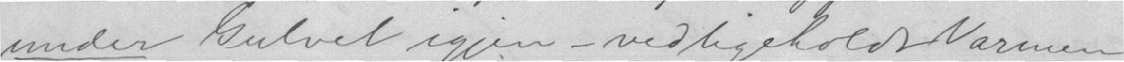under Gulvet igjen - vedligehold Varmen
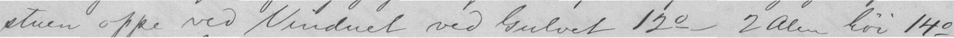stuen oppe ved Vinduet ved Gulvet 12. 2 Alen høi 14.
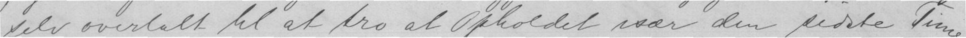selv overtalt til at tro at Opholdet især den sidste Time
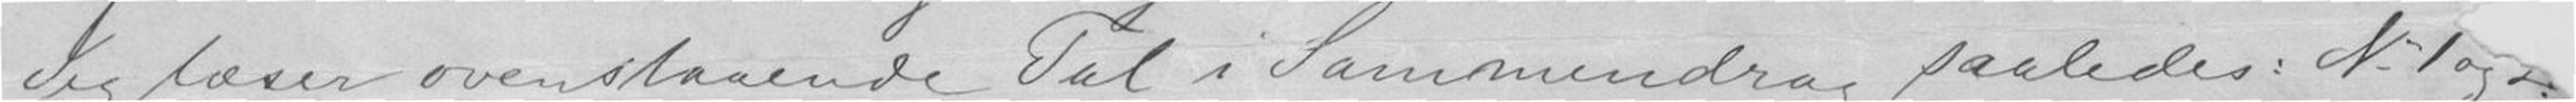Jeg læser overstaaende Tal i Sammendrag saaledes: N.1 og 2Bit borte
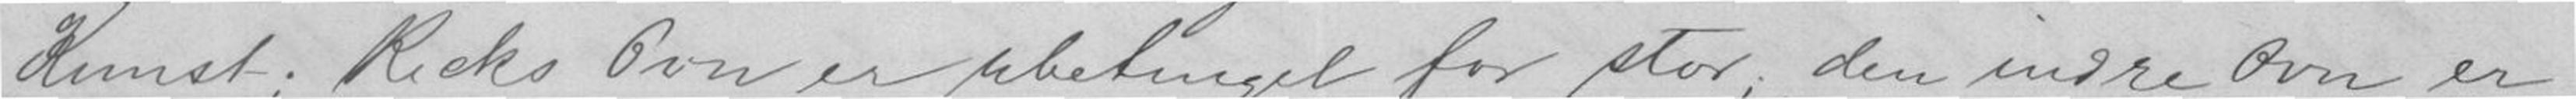Kunst; Recks Ovn er ubetinget for stor; den indre Ovn er
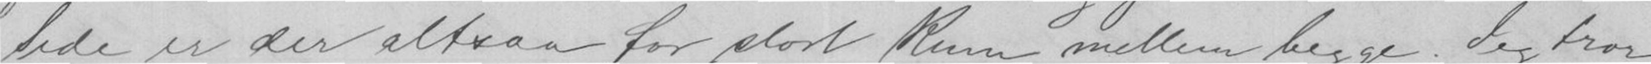Side er der altsaa for stort Rum mellem begge. Jeg tror
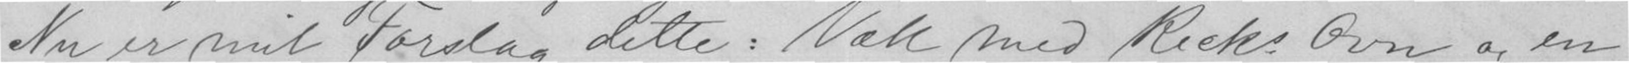Nu er mit Forslag dette: Væk med Recks Ovn og en
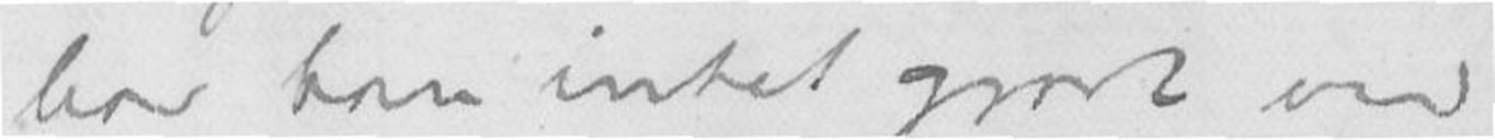har han intet gjort ved
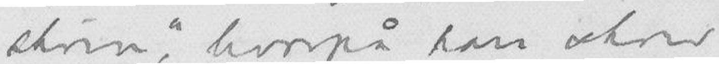skrive", hvorpå han skrev
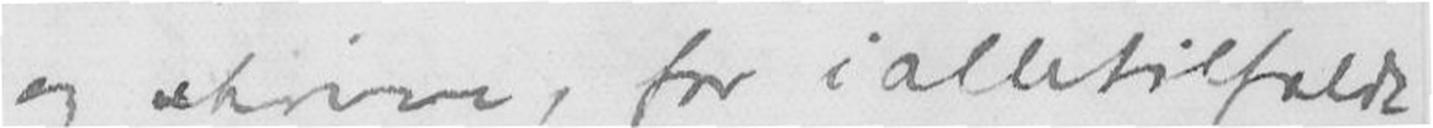og skrive, for ialletilfælde
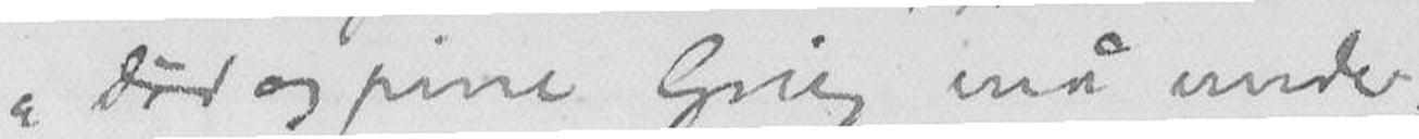"død og pine Grieg må under-
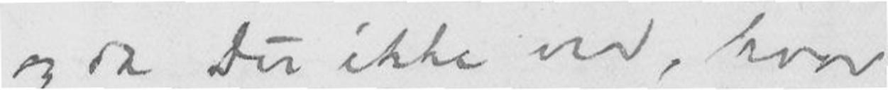og da Du ikke ved, hvor
